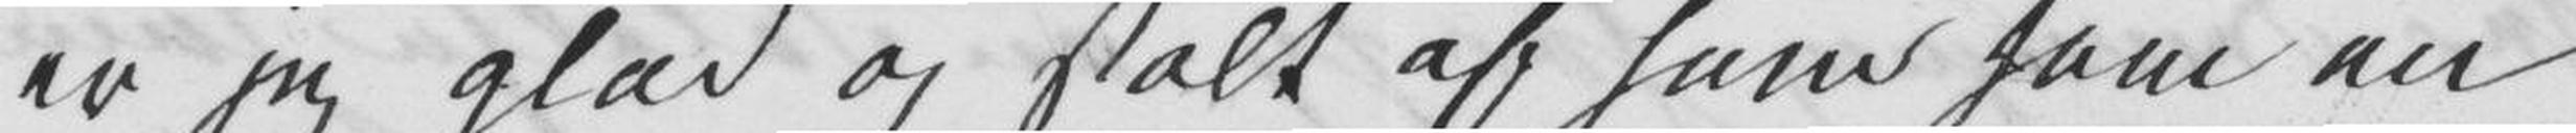er jeg glad og stolt af ham som en
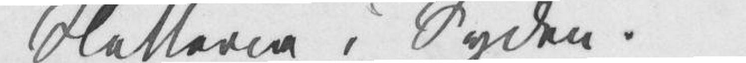Sletterne i Syden.
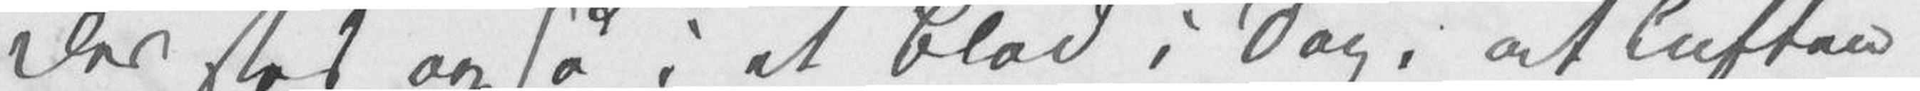Der stod også i et Blad i Dag, at Luften
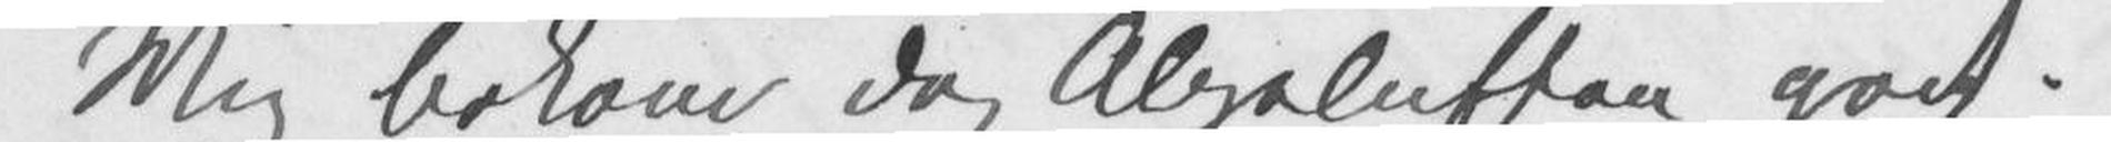Mig bekom dog Alpeluften godt.
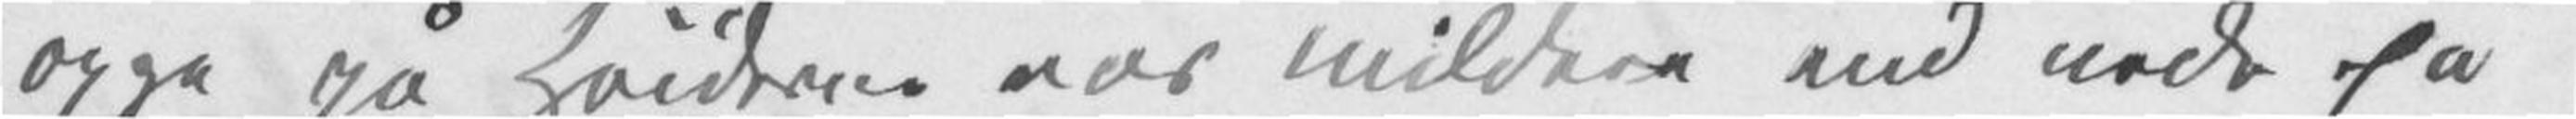oppe på Høiderne var mildere end nede på
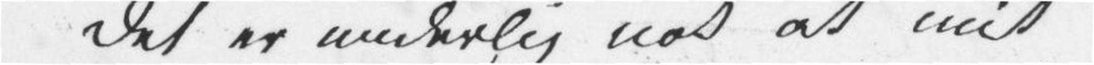Det er underlig nok at mit
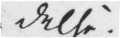delse.
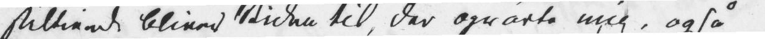stiltiende bliver Vidne til, der oprørte mig, og så
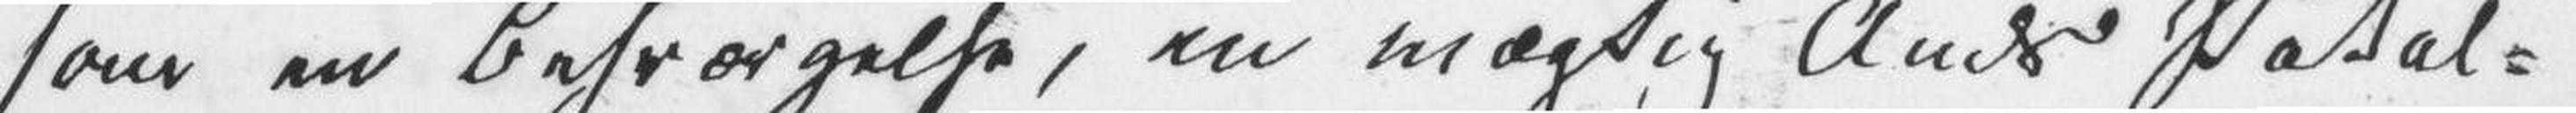som en Besværgelse, en mægtig Ånds Påkal¬
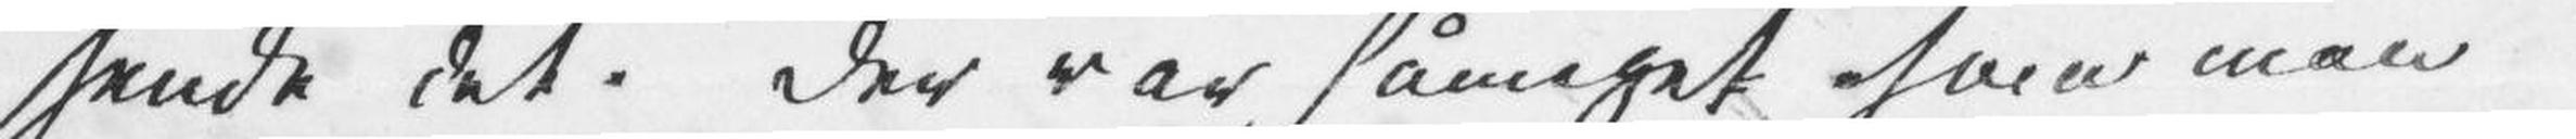sende det. Der var såmeget som man
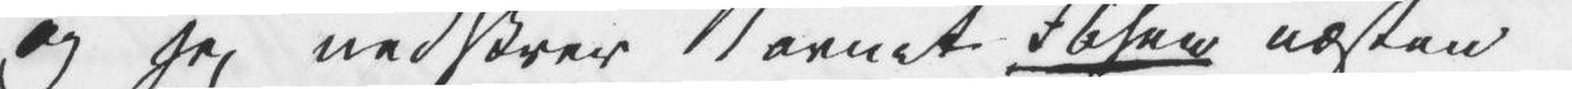og jeg nedskrev Navnet Ibsen næsten
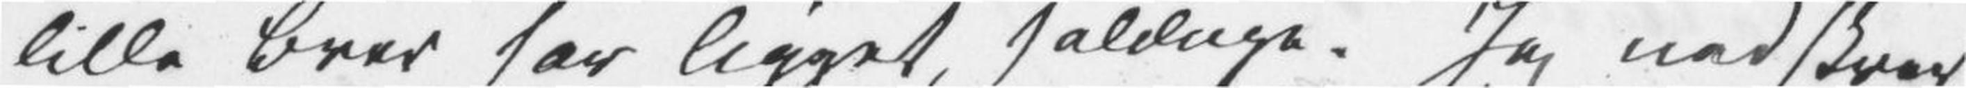lille Brev har ligget sålænge. Jeg nedskrev
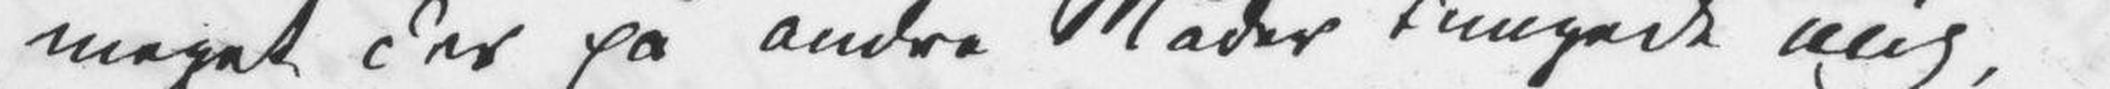meget der på andre Måder knugede mig,
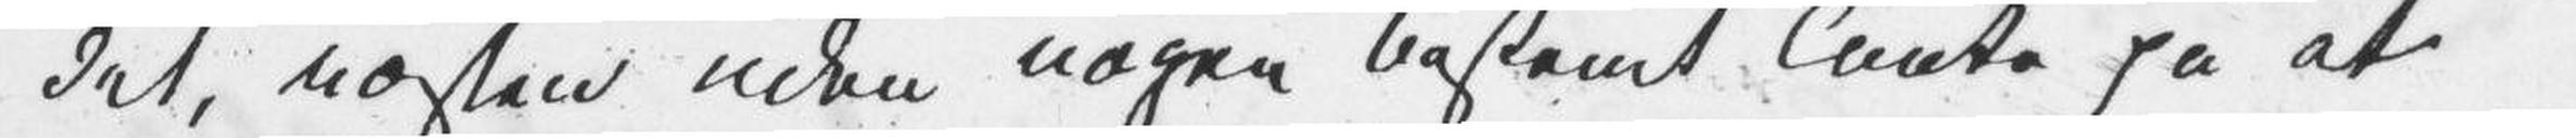det, næsten uden nogen bestemt Tanke på at
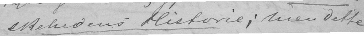skehedens Historie; men dette
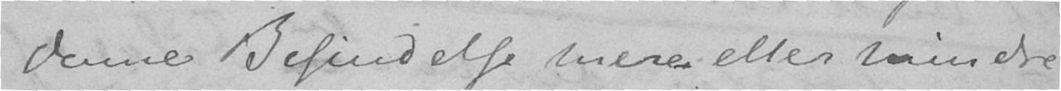denne Befindelse mere eller mindre
